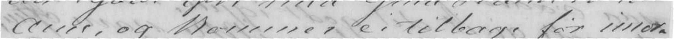Arne, og kommer ei tilbage før imor-
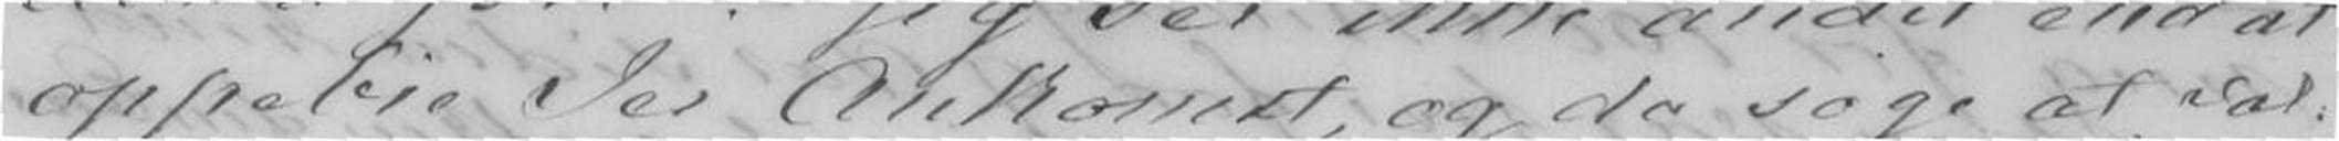oppebie Jer Ankomst, og da søge at væl-
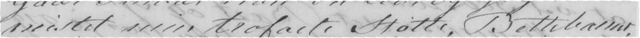mistet min trofaste Støtte, Bettebarnet,
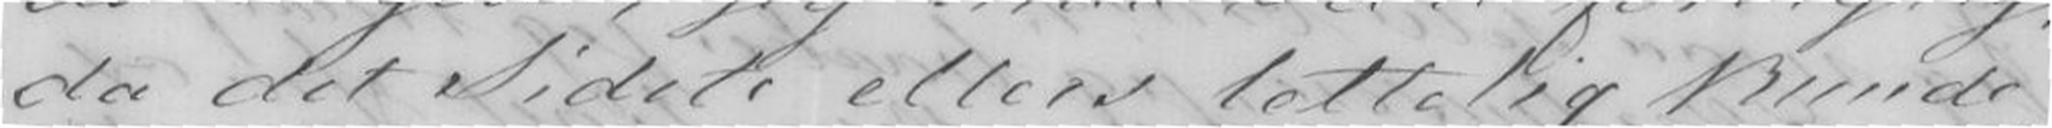da det Sidste ellers lettelig kunde
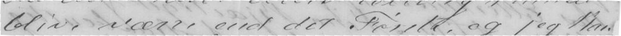blive værre end det Første, og jeg kan
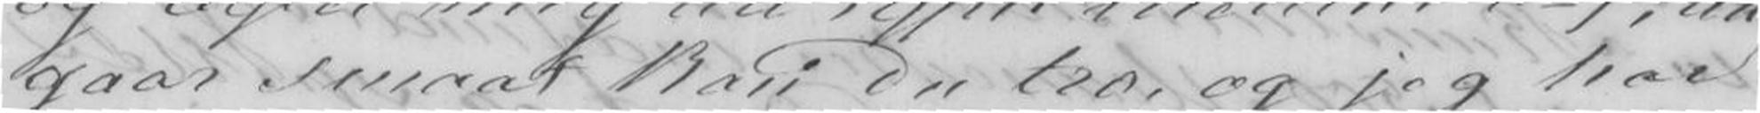gaar smaat kan Du tro, og jeg har
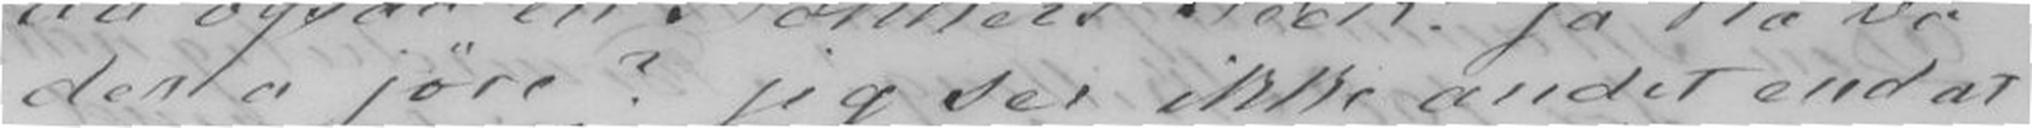der a jøre? jeg ser ikke andet end at
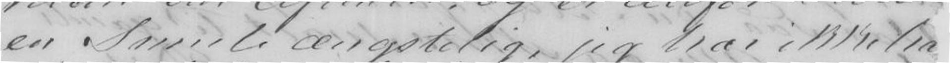en Smule ængstelig, jeg har ikke ha
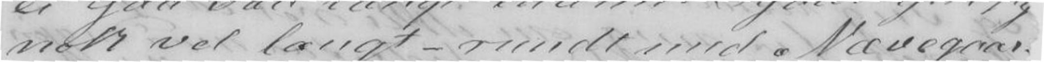nok vel langt - rundt med Nævegaar-
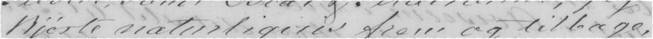kjørte naturligviis frem og tilbage,
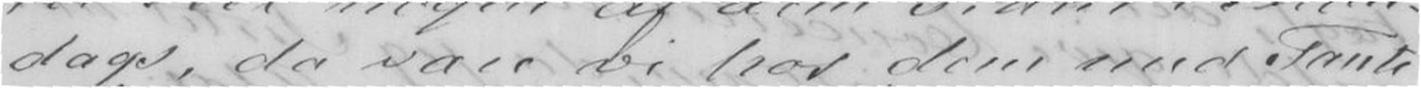dags, da vare vi hos dem med Tante
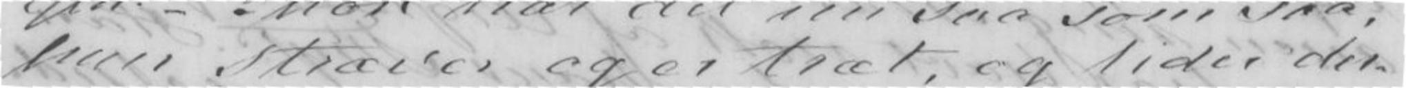hun stræver og er træt, og lider der-
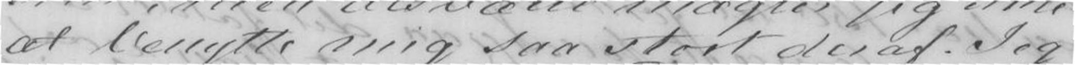at benytte mig saa stort deraf. Jeg
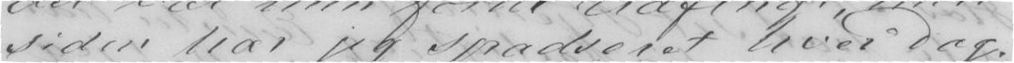siden har jeg spadseret hver Dag.
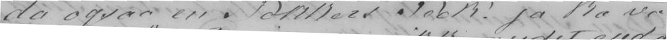da ogsaa en Pokker Peek"! ja ka va
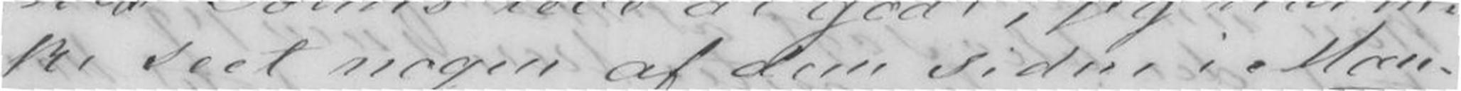ke seet nogen af dem siden i Man-
